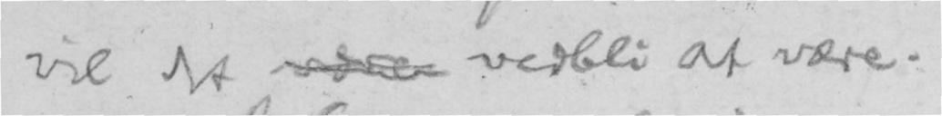vil det være vedbli at være.
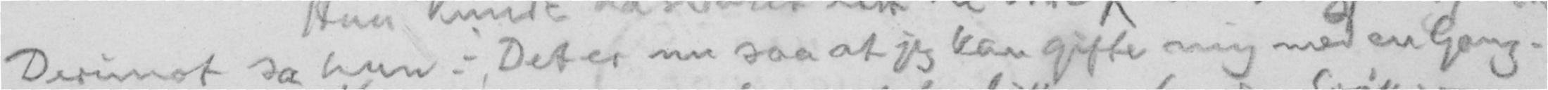Derimot sa hun: Det er nu saa at jeg kan gifte mig med en Gang.
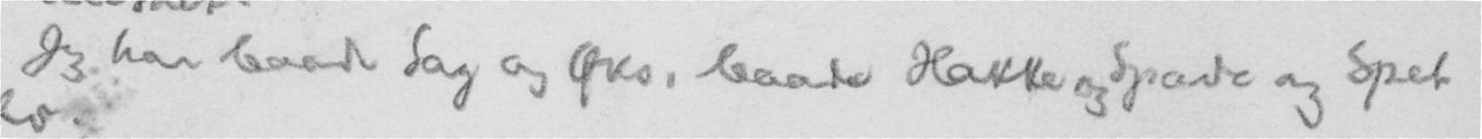Jeg har baade Sag og Øks, baade Hakke og Spade og Spet
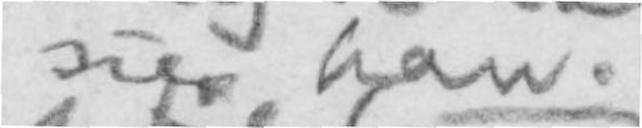sier han.
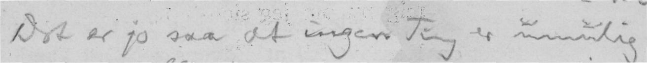Det er jo saa at ingen Ting er umulig
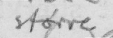større
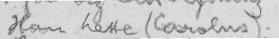Han hette (Carolus).
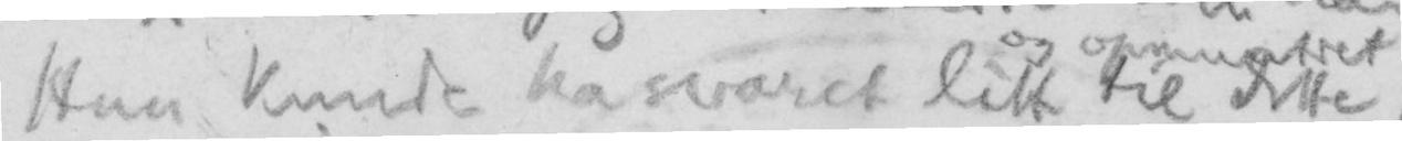Hun kunde ha svaret litt til dette
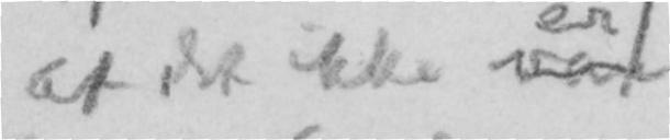at det ikke var
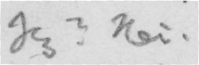Jeg? Nei.
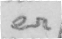er
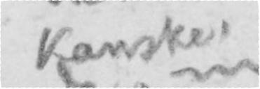kanske,
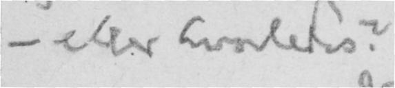- eller hvorledes?
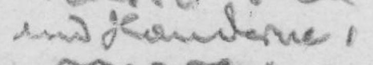end Hænderne,
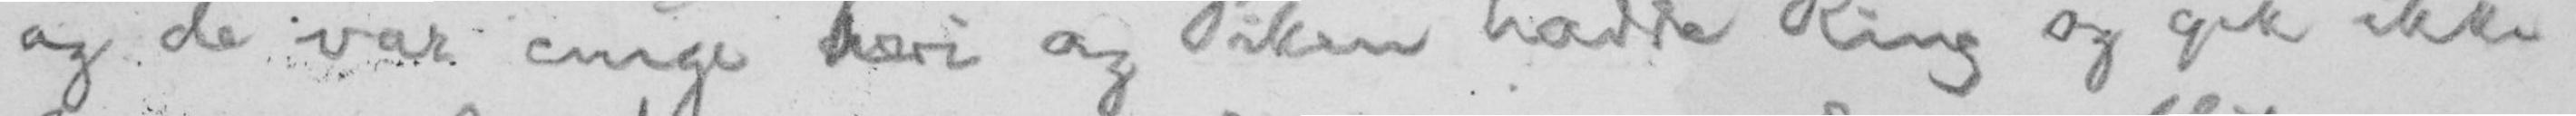og de var enige heri og Piken hadde Ring og gik ikke
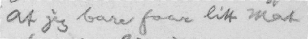at jeg bare faar litt Mat
